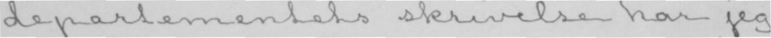departementets skrivelse har jeg
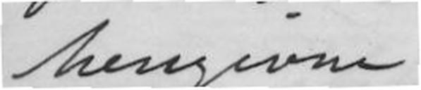hengivne
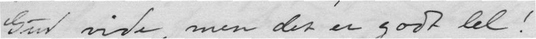Gud vide, men det er godt lel!
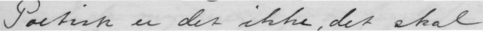Poetisk er det ikke, det skal
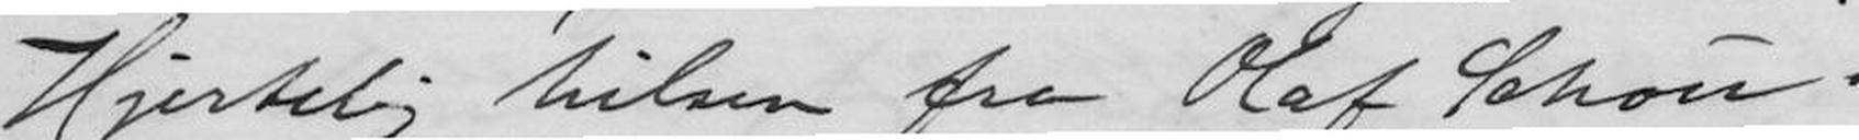Hjertelig hilsen fra Olaf Schou,
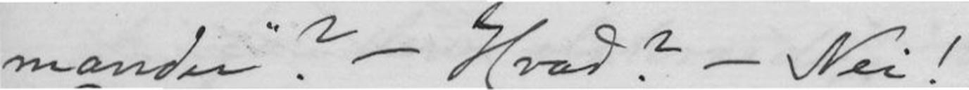mander? - Hvad? - Nei!
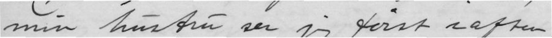min hustru ser jeg først iaften,
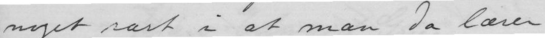noget rart i at man da lærer
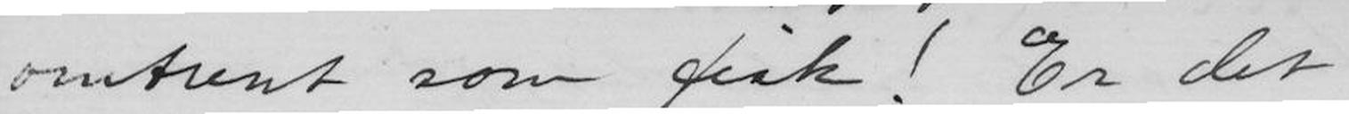omtrent som fisk! Er det
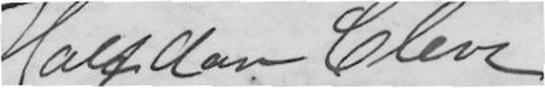Halfdan Cleve
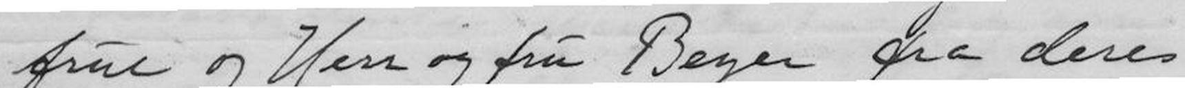frue og Herr og fru Beyer fra dres
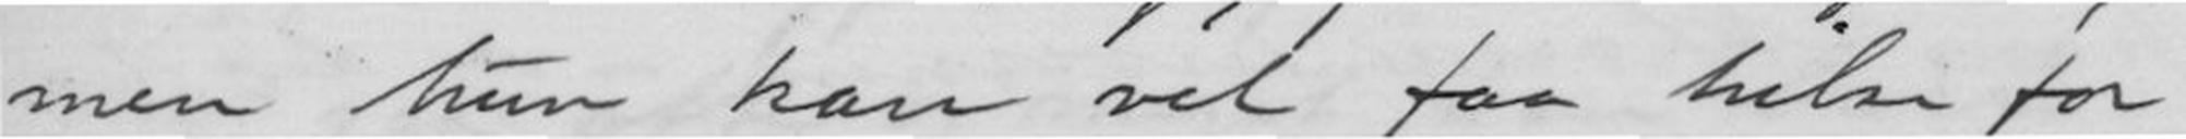men hun kan vel faa hilse for
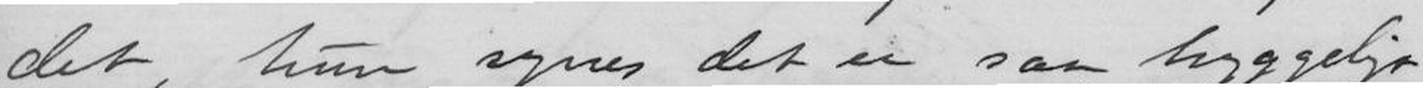det, hun synes det er saa hyggeligt
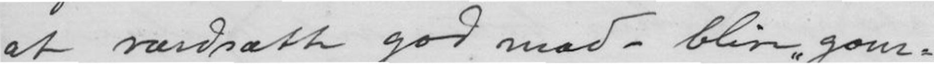at værdsætte god mad - blive gour-
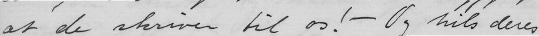at de skriver til os! - Og hils deres
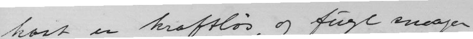kost er kraftløs, og fugl smager
